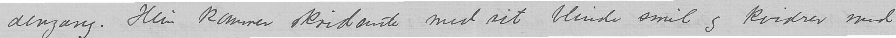dengang. Hun kommer skridende med sit blinde smil og kvidrer med
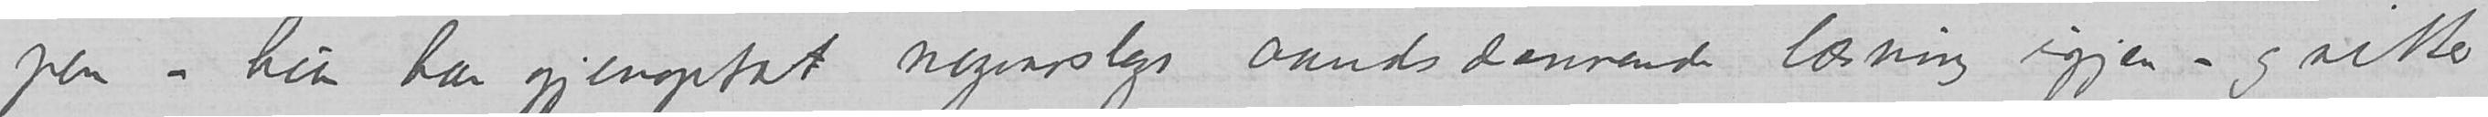pen – hun har gjenoptat nogenslags aandsdannende læsning igjen – og sitter
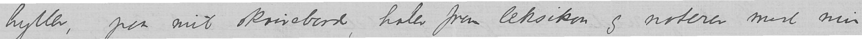hyller, paa mit skrivebord, haler frem leksikon og noterer med min
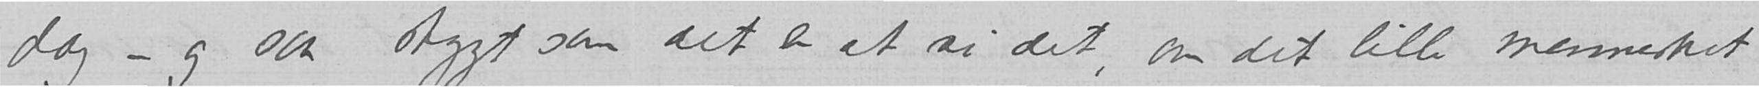dag – og saa stygt som det er at si det, om det lille mennesket
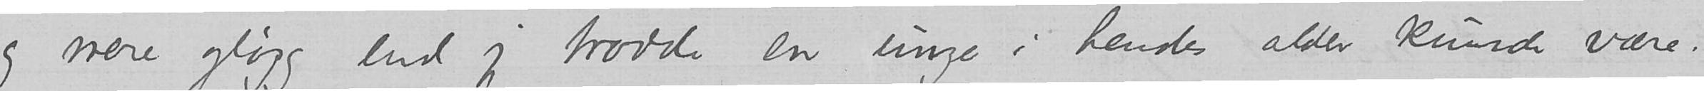og mere gløgg end jeg trodde en unge i hendes alder kunde være.
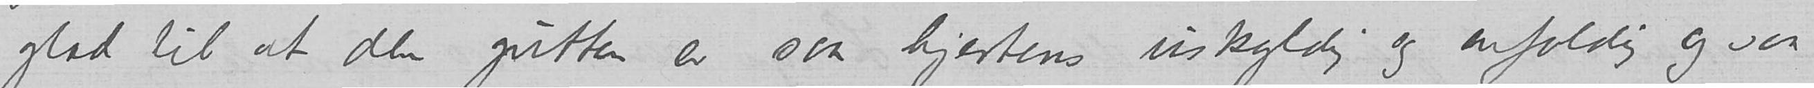glad til at den gutten er saa hjertens uskyldig og enfoldig og saa
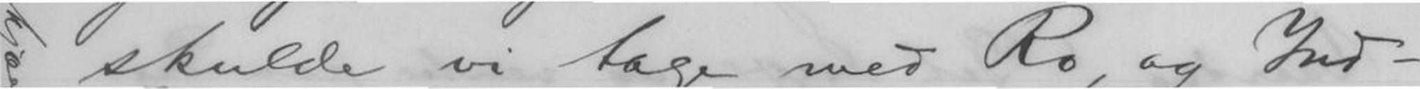skulde vi tage med Ro, og Ind-
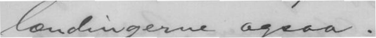lændingerne ogsaa. -
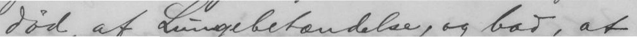død, af Lungebetændelse, og bad, at
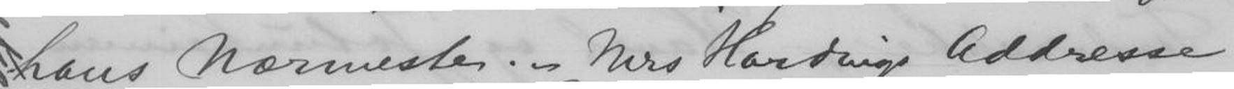hans Nærmeste. - Mrs Hardings Addresse
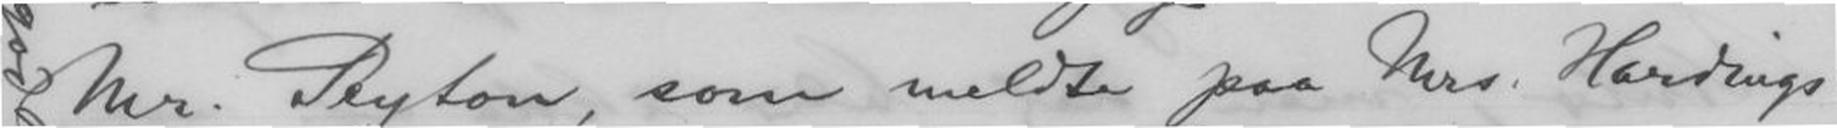Mr. Peyton, som meldte paa Mrs. Hardings
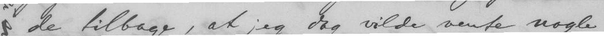de tilbage, at jeg dog vilde vente nogle
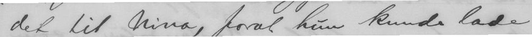det til Nina, forat hun kunde lade
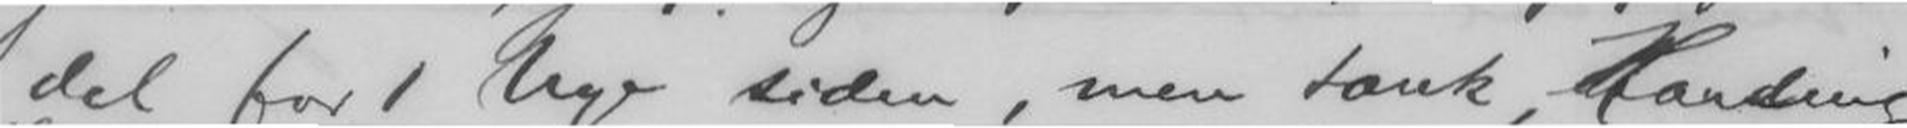høre det for 1 Uge siden, men tænk, Harding
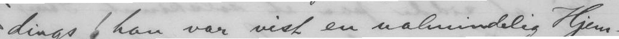dings! han var vist en ualmindelig Hjem-
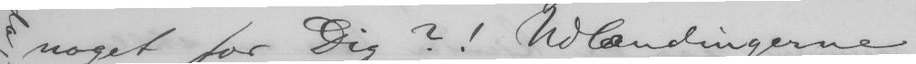noget for Dig?! Udlændingerne
